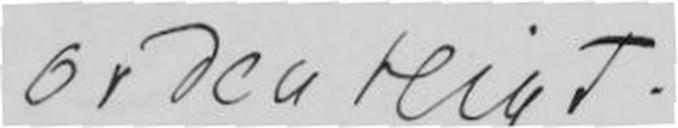ordentligt.
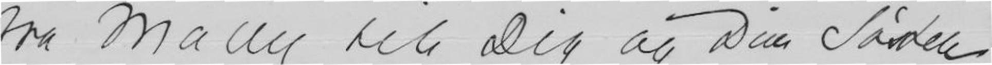fra Mally til Dig og Din Sødster
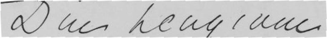Din hengivne
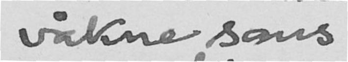våkne sans
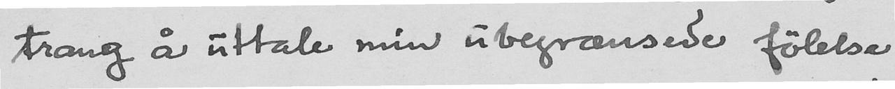trang å uttale min ubegrænsede følelse
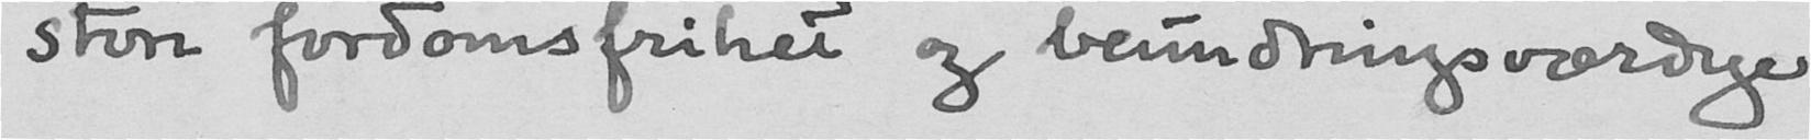store fordomsfrihet og beundringsværdige
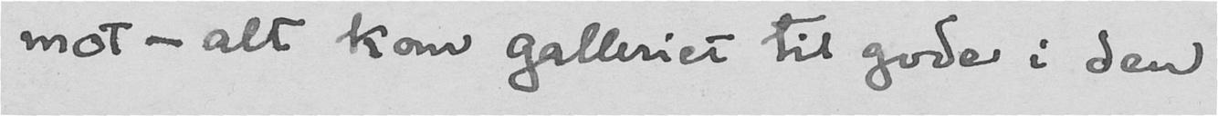mot - alt kom galleriet til gode i den
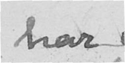har
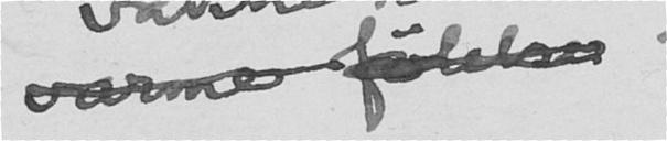varme følelser
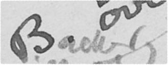Backer
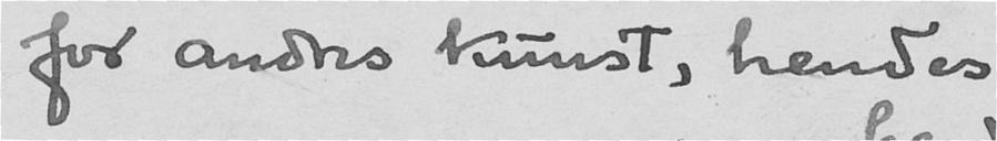for andres kunst, hendes
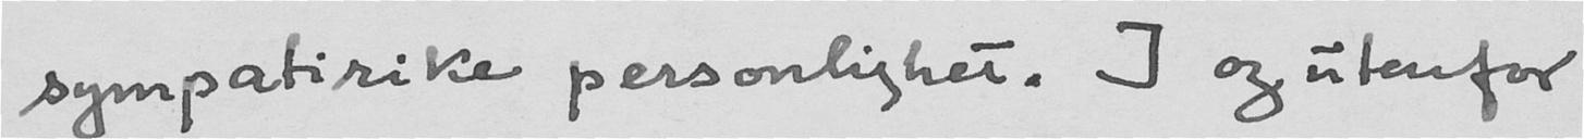sympatirike personlighet. I og utenfor
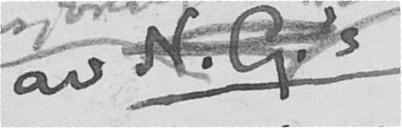av N.G.'s
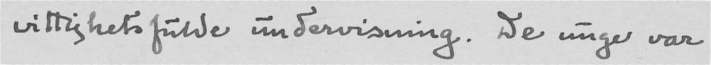vittlighetsfulde undervisning. De unge var
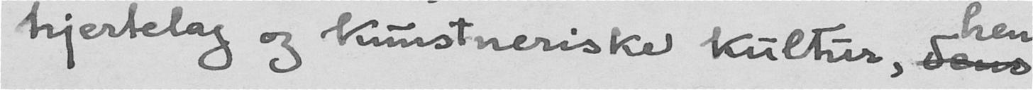hjertelag og kunstneriske kultur, dens
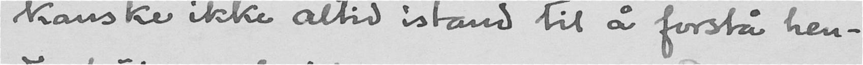kanske ikke altid istand til a forstå hen-
